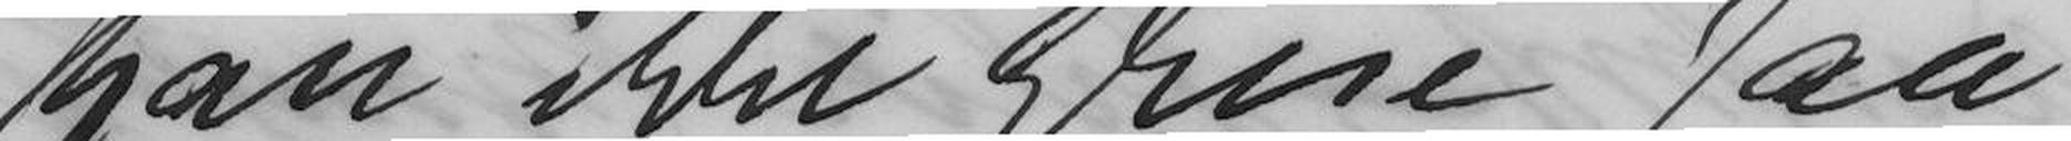kan ikke greie saa
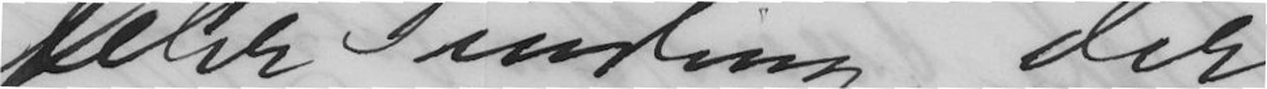Dhr Sinding der
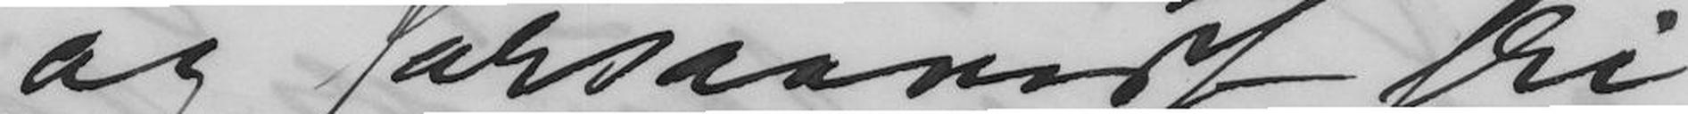og forsaavidt fri
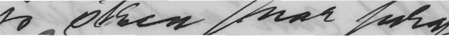jo strax var forat,
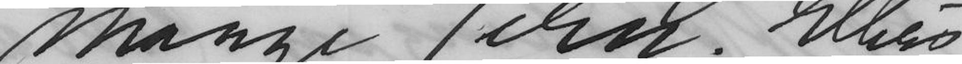mange Jern. Ellers
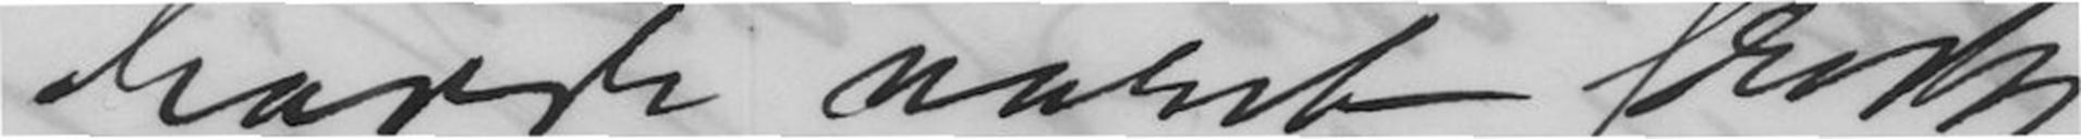havde været frisk
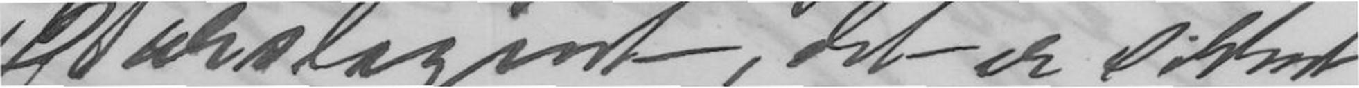Storslagent, det er sikkert.
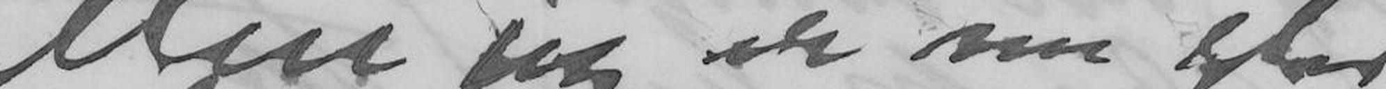Men jeg er nu glad
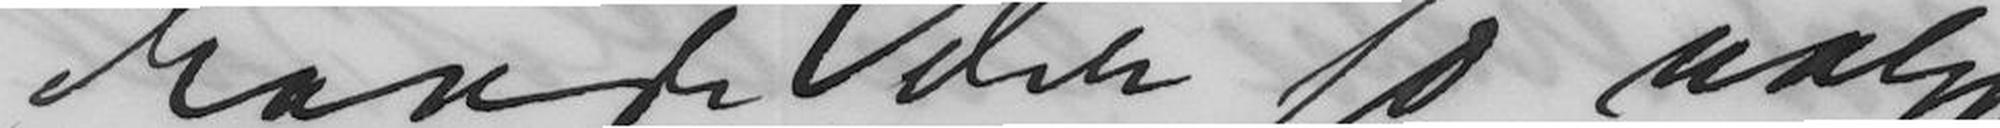havde Den jo været
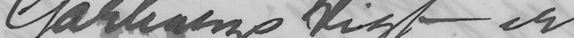Garborgs Digt er
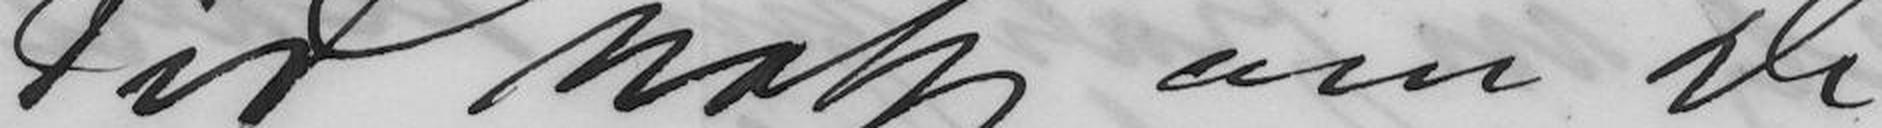Tid nok om De
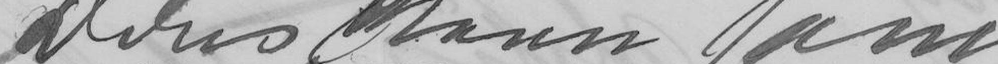Deres Navn som
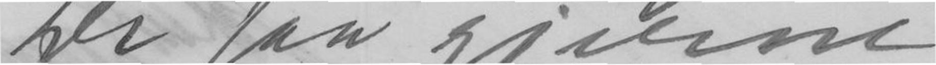De saa gjerne
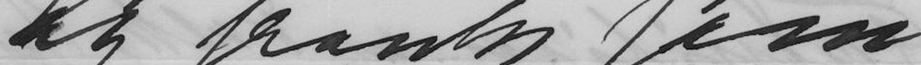og frank som
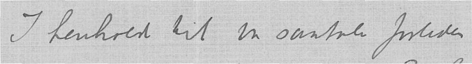I henhold til vor samtale forleden
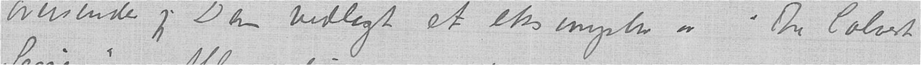oversender jeg Dem vedlagt et eksemplar av «The Calvert
